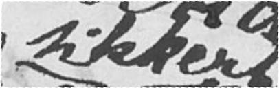sikkert
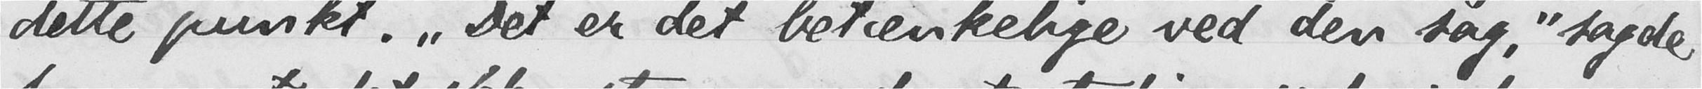dette punkt. "Det er det betænkelige ved den sag," sagde
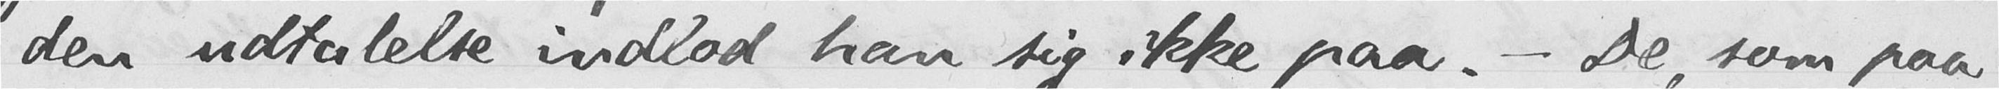den udtalelse indlod han sig ikke paa. - De, som paa
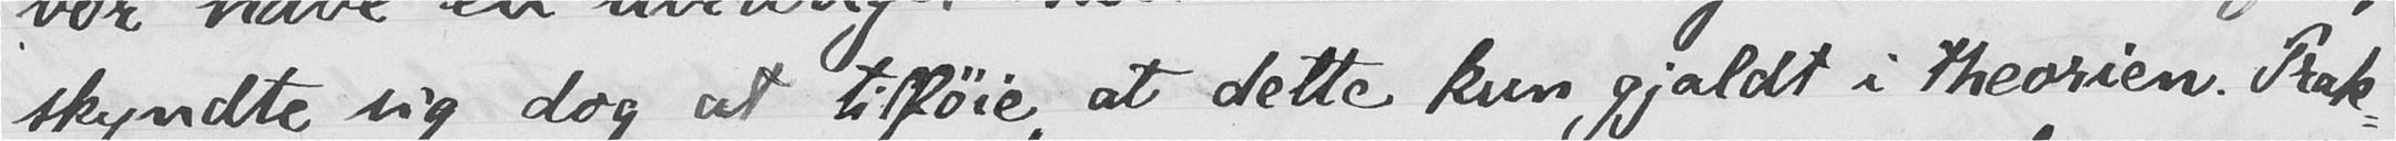skyndte sig dog at tilføie, at dette kun gjaldt i theorien. Prak-
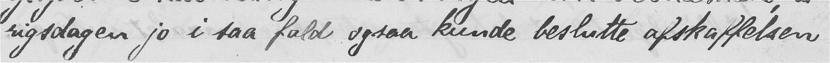rigsdagen jo i saa fald ogsaa kunde beslutte afskaffelsen
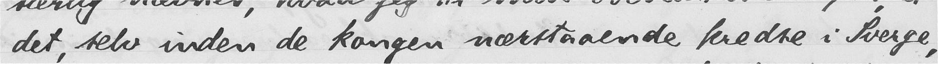det, selv inden de kongen nærstaaende kredse i Sverge,
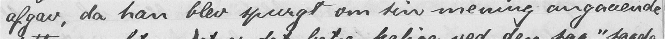afgav, da han blev spurgt om sin mening angaaende
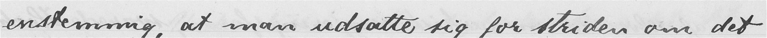enstemmig, at man udsatte sig for striden om det
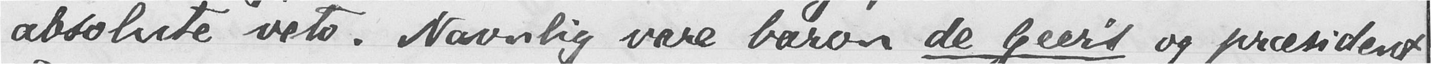absolute veto. Navnlig vare baron de Geer's og præsident
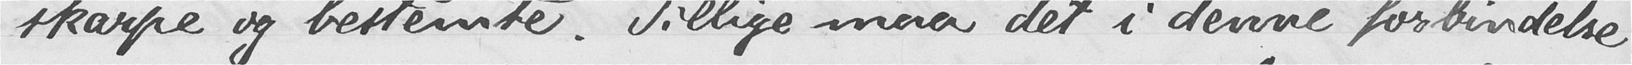skarpe og bestemte. Tillige maa det i denne forbindelse
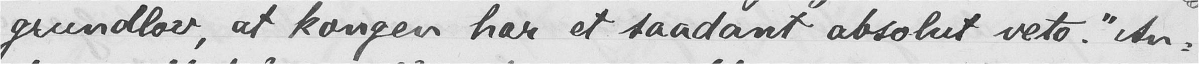grundlov, at kongen har et saadant absolut veto." An-
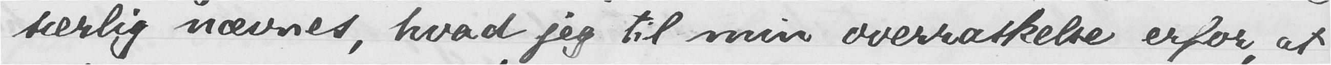særlig nævnes, hvad jeg til min overraskelse erfor, at
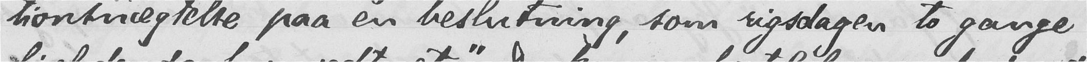tionsnægtelse paa en beslutning, som rigsdagen to gange
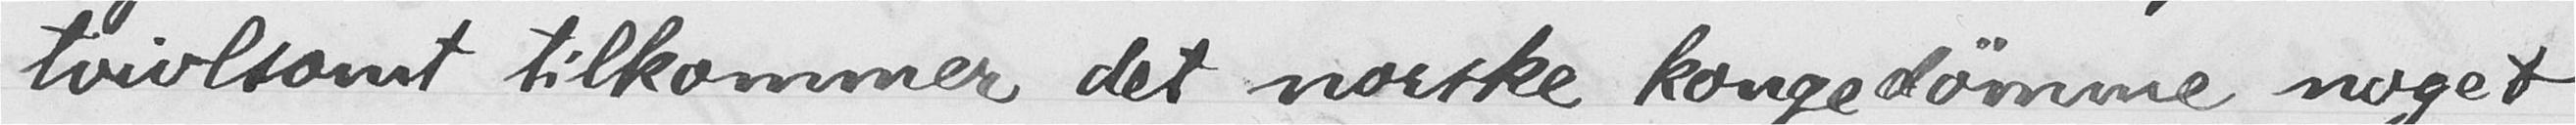tvivlsomt tilkommer det norske kongedømme noget
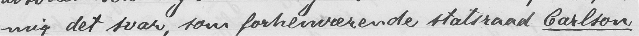mig det svar, som forhenværende statsraad Carlson
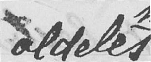aldeles
